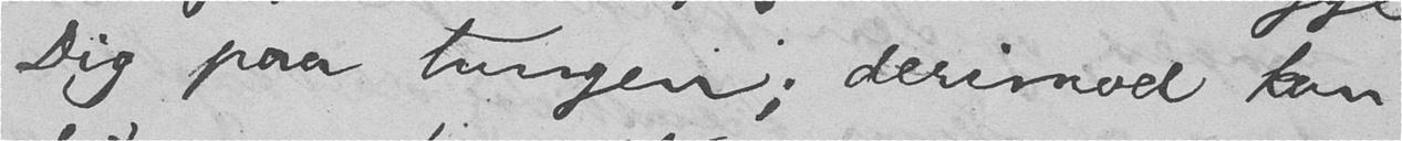Dig paa tungen; derimod kan
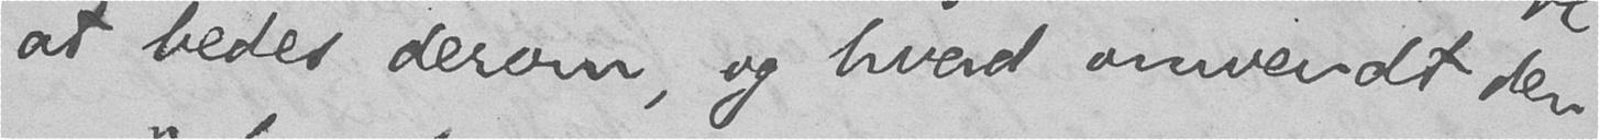at bedes derom, og hvad omvendt den
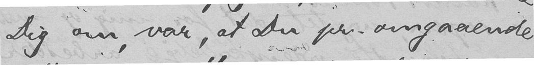Dig om, var, at Du pr. omgaaende
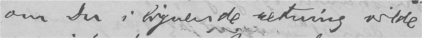om Du i lignende retning vilde
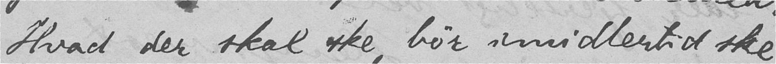Hvad der skal ske, bør imidlertid ske
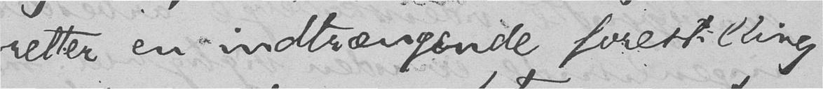retter en indtrængende forestilling
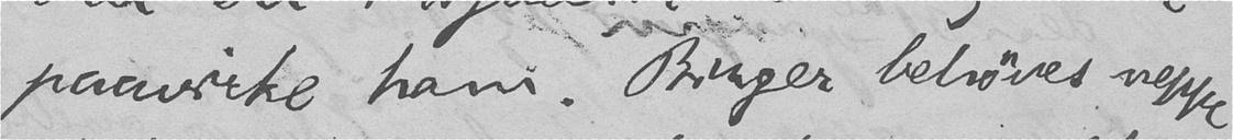paavirke ham. Birger behøves neppe
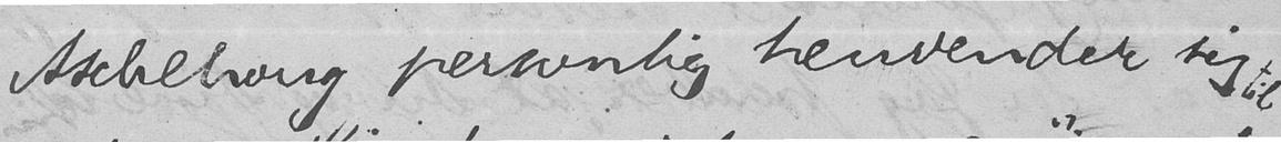Aschehoug personlig henvender sig til
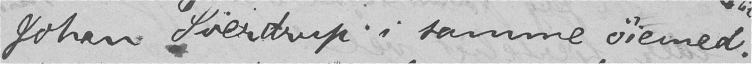Johan Sverdrup i samme øiemed.
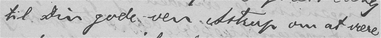til Din gode ven Astrup om at være
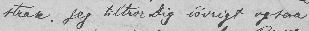strax. Jeg tiltror Dig iøvrigt ogsaa
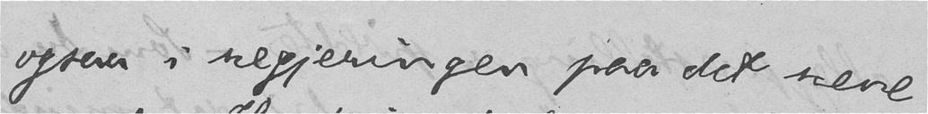ogsaa i regjeringen paa det rene
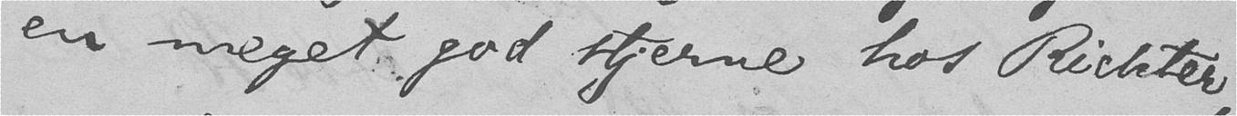en meget god stjerne hos Richter,
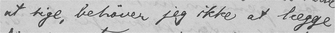at sige, behøver jeg ikke at lægge
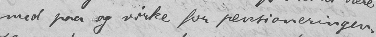med paa og virke for pensioneringen.
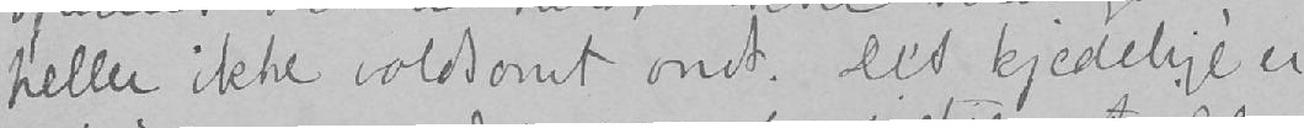heller ikke voldsomt ondt. Det kjedelige er,
